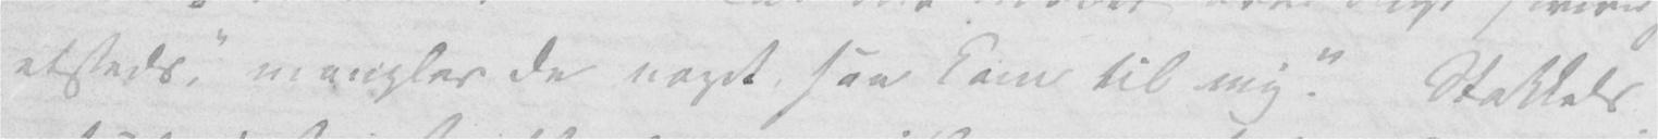etsteds, «mangler De noget, saa kom til mig.» Stakkels
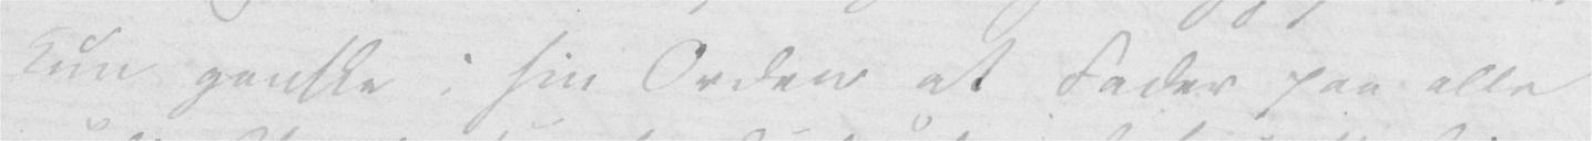kun ganske i sin Orden at Fader paa alle
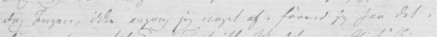dog Ingen, ikke engang jeg noget af. førend jeg saa det i
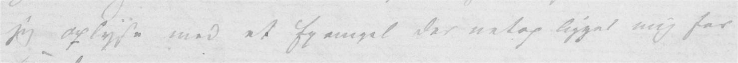jeg oplyse med et Exempel der netop ligger mig for
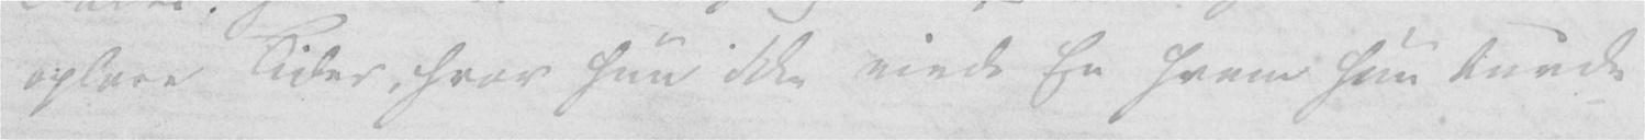opleve Tider, hvor hun ikke eiede En hvem hun kunde
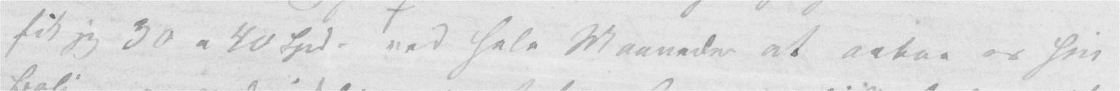fik jeg 30 a 40 Spd. ved hele Maaneder at aabne os sin
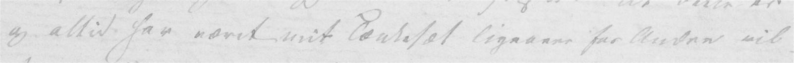og altid har været mit Tænkesæt ligeover for Andre vil
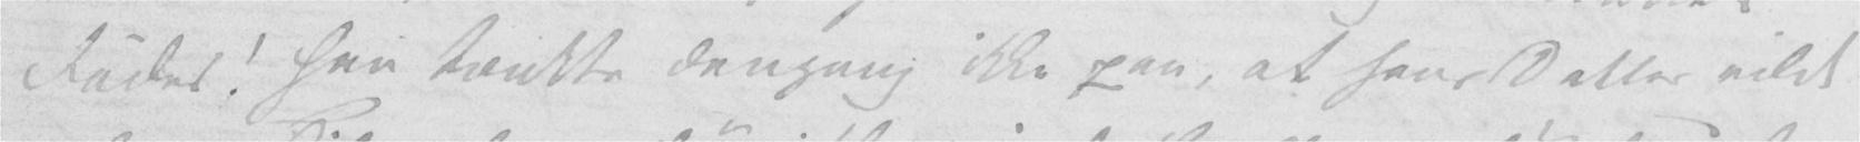Fader! han tænkte dengang ikke paa, at hans Datter vilde
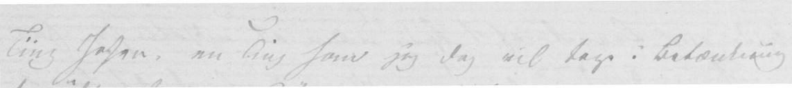Ting Johan, en Ting som jeg dog vil tage i Betænkning
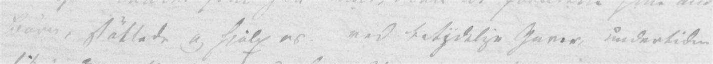Børn, støttede og hjalp os ved betydelige Gaver, undertiden
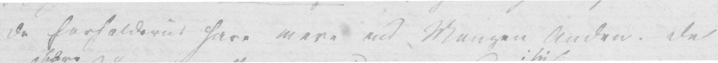de forholdsviis have mere end Mangen Anden. De
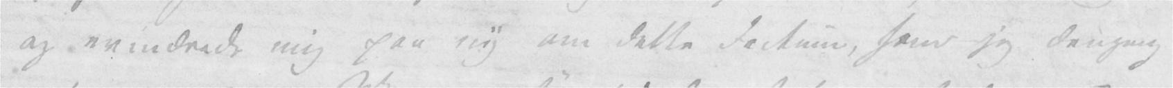og erindrede mig paa ny om dette Factum, som jeg dengang
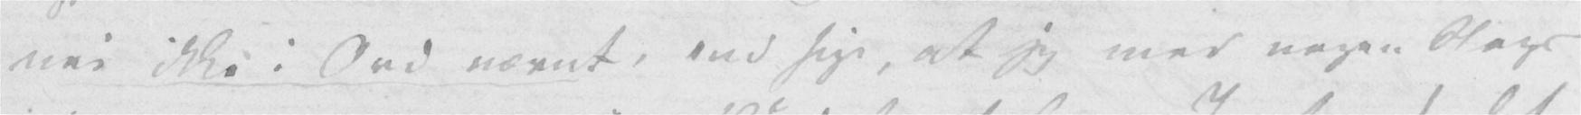nei ikke i Ord nævnt, end sige, at jeg med nogen Slags
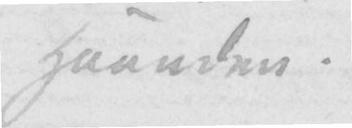Haanden.
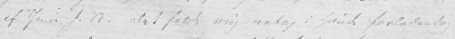af Juni s. A. Det faldt mig netop i Hænde forledendag
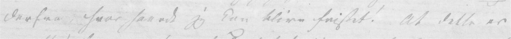derfra, hvor haardt jeg kan blive fristet! At dette er
